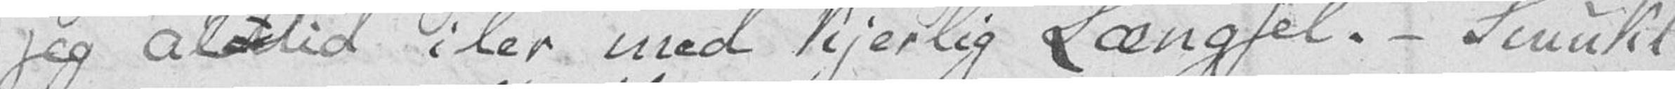jeg aldtid iler med kjerlig Længsel. – Smukt
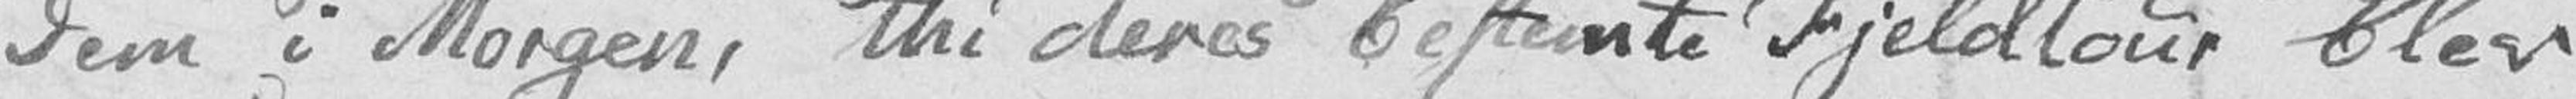Dem i Morgen, thi deres bestemte Fjeldtour blev
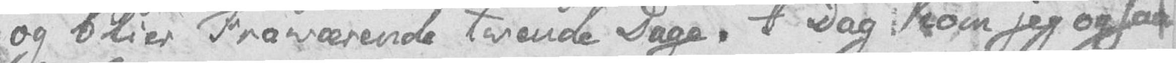og blier Fraværende tvende Dage. I Dag kom jeg ogsaa
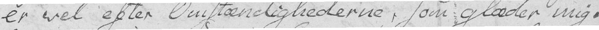er vel efter Omstændighederne, som glæder mig.
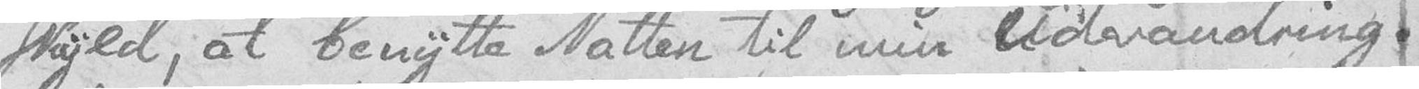skyld, at benytte Natten til min Udvandring.
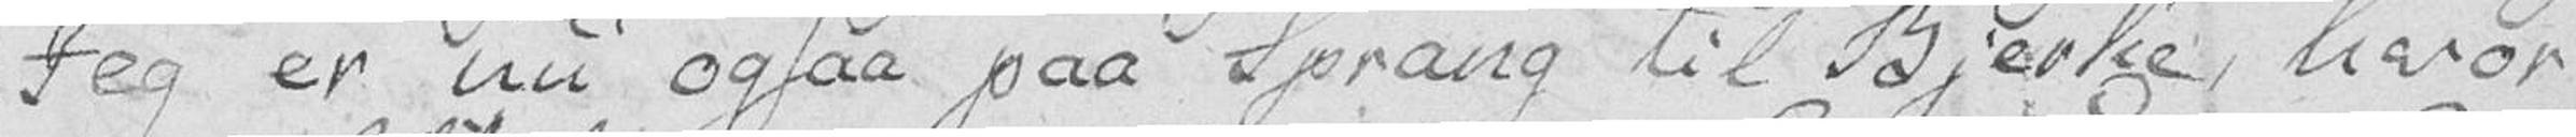Jeg er nu ogsaa paa Sprang til Bjerke, hvor
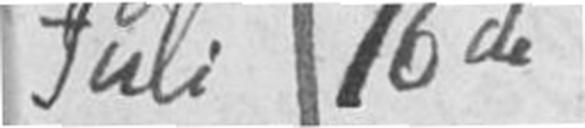Juli 16de
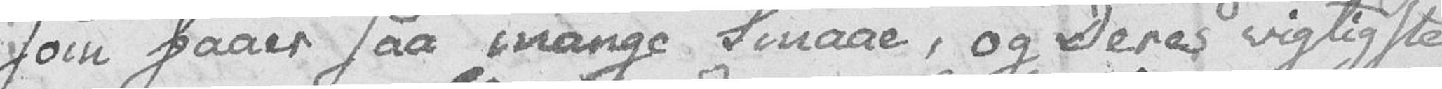som faaer saa mange Smaae, og Deres vigtigste
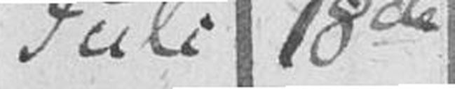Juli 18de
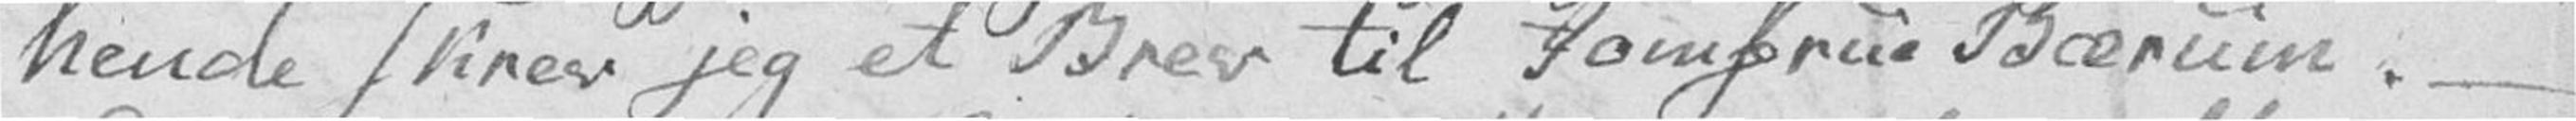hende skrev jeg et Brev til Jomfrue Bærum. –
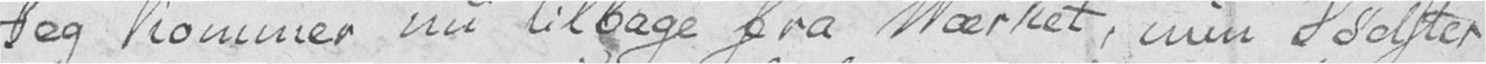Jeg kommer nu tilbage fra Værket, min Sødster
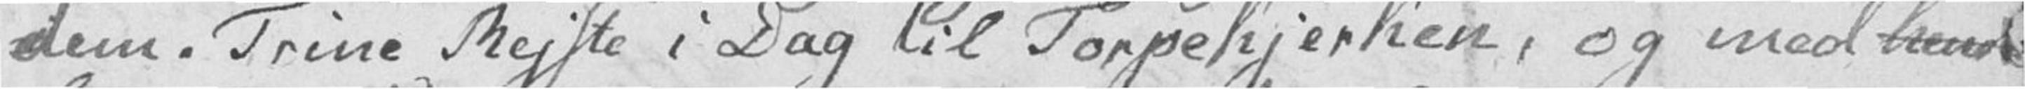dem. Trine Rejste i Dag til Torpekjerken, og med hende
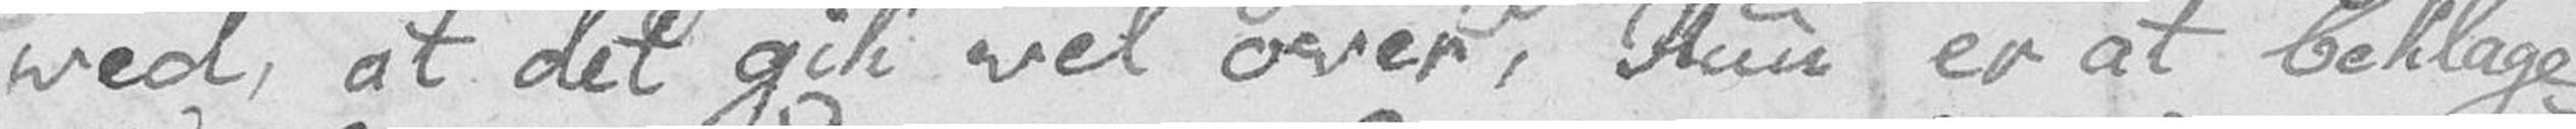ved, at det gik vel over, Hun er at beklage
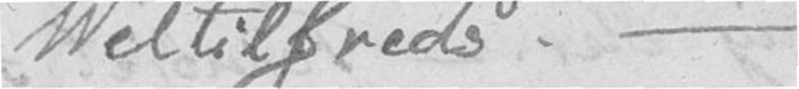Veltilfreds. –
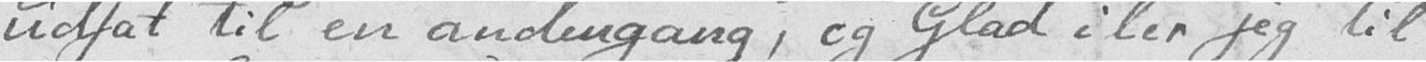udsat til en andengang, og Glad iler jeg til
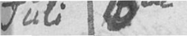Juli 15de
